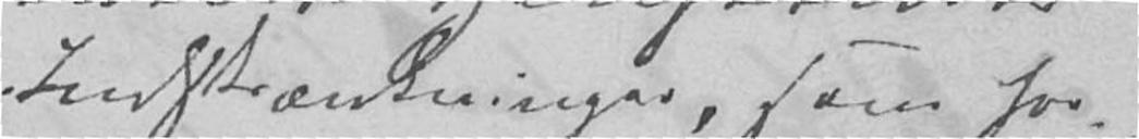Indskrænkninger, som for-
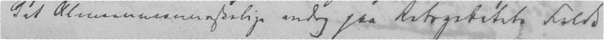det Almeenmenneskelige endog paa Retsgebetets Feldt
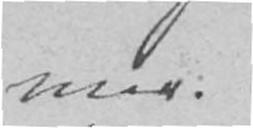mer.
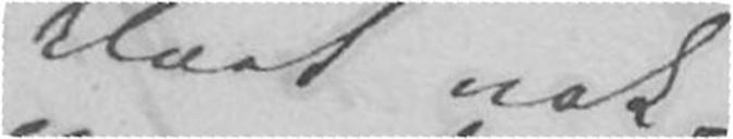klart nok
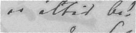er altid be-
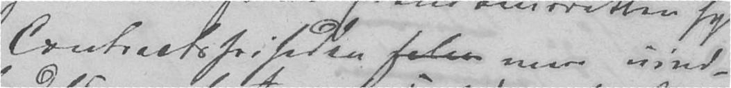Contractsfriheden mere uind-
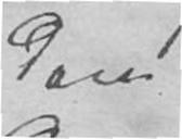dom-
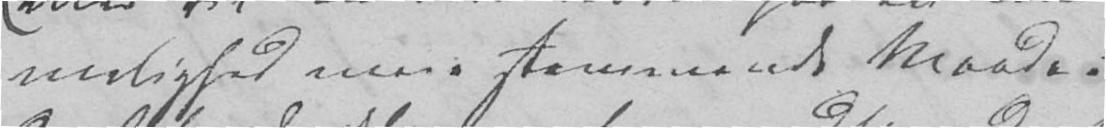melighed mere stemmende Maade.
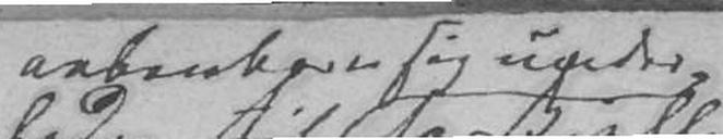abenbarer sig under
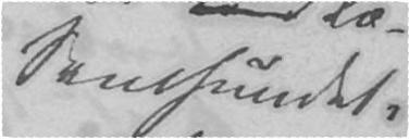Samfundets
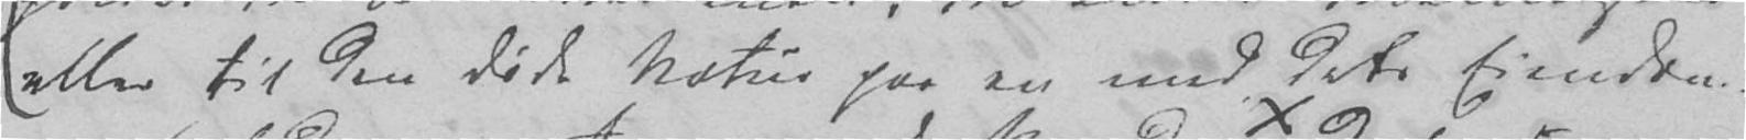eller til den døde Natur paa en med dets Eiendom-
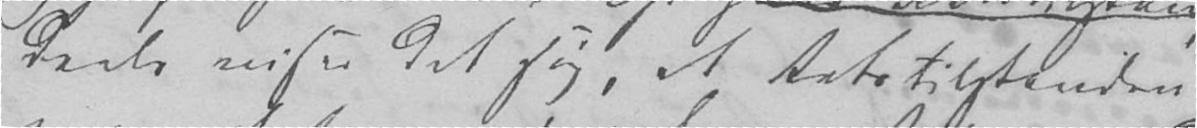deels viser det sig, at Retstilstanden
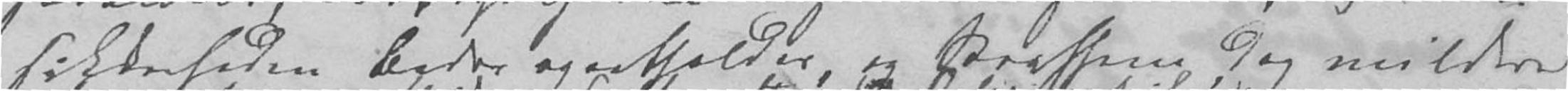sikkerheden bedre opretholdes , og Straffene dog mildere
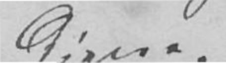digere,
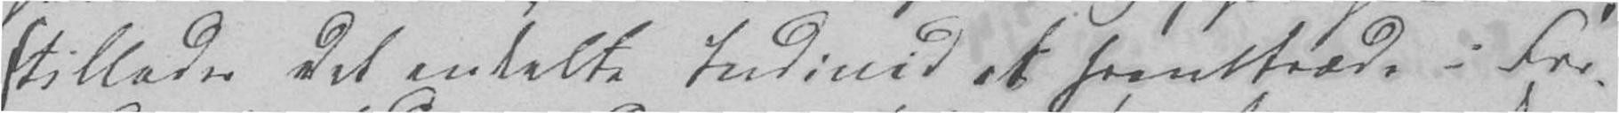tillader det enkelte Individ at framtræde i For-
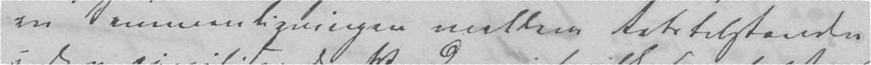en Sammenligningen mellem Retstilstanden
